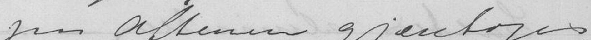paa Aftenen gjæntages
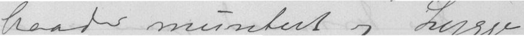baade muntert og hygge
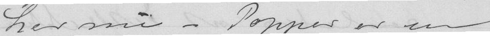her nu - Popper er en
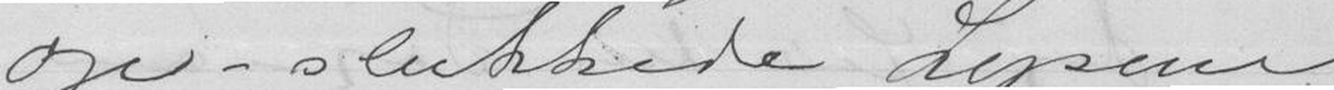op - slukkede Lyserne
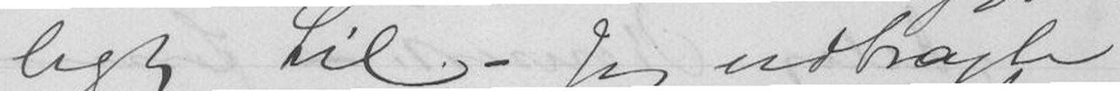ligt til. Jeg udbragte
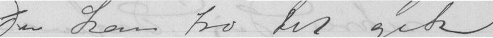Du kan tro det gik
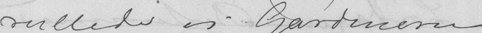rullede vi Gardinerne
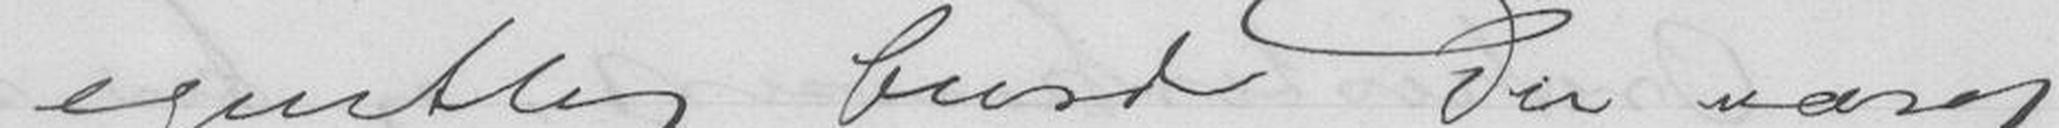egentlig burde Du været
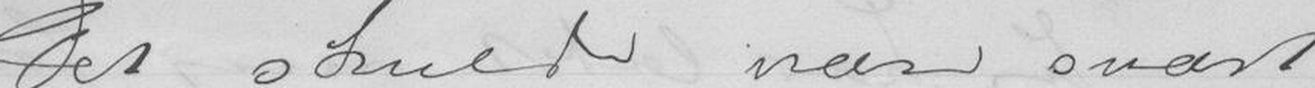Det skulde være svært
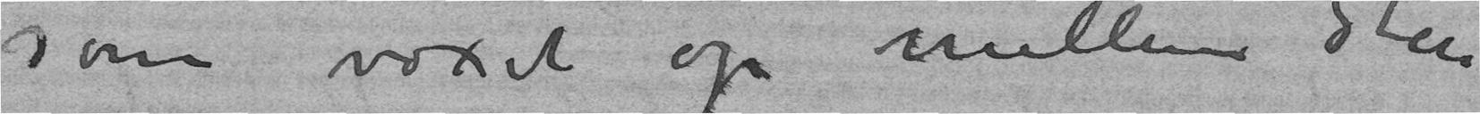som har voxet op mellem Sten
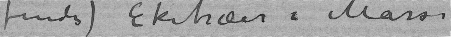findes) Eketræer i Masser
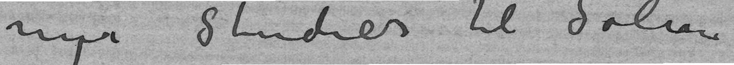nye Studier til Solen
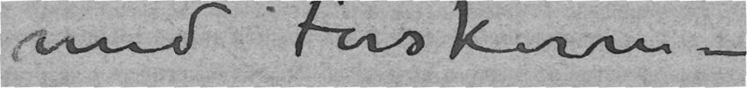med Forskerne –
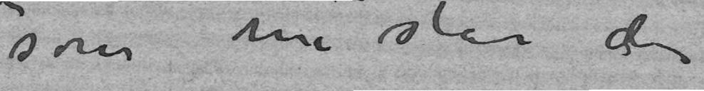som nu står der
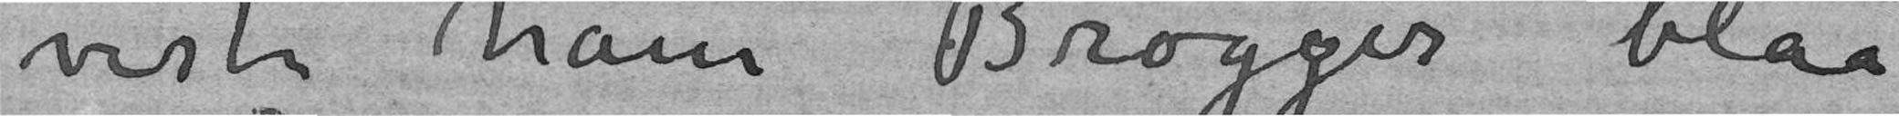viste ham Brøggers blaa
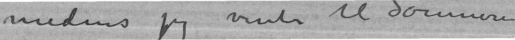medens jeg venter til Sommeren
